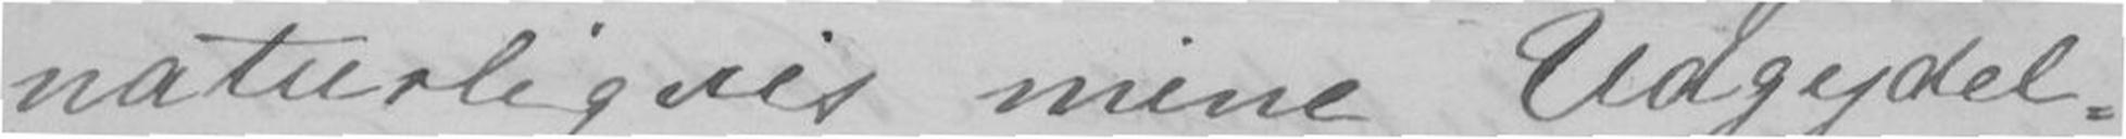naturligvis mine Udgydel-
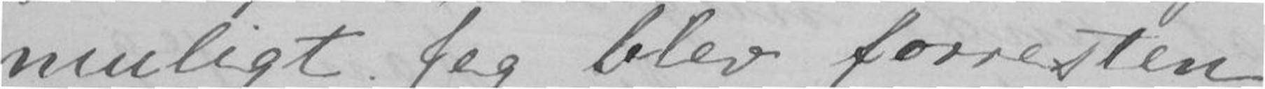muligt. Jeg blev forresten
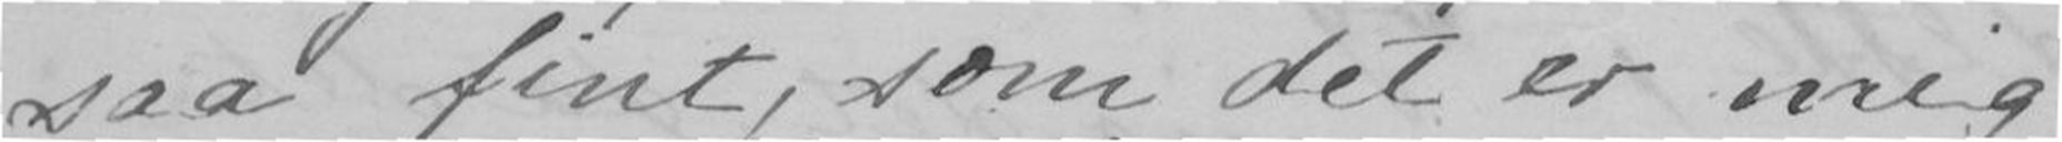saa fint, som det er mig
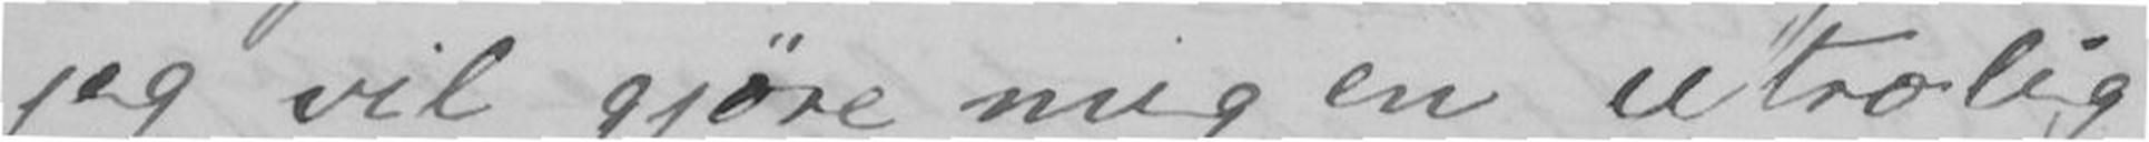jeg vil gjøre mig en utrolig
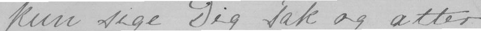kun sige Dig Tak og atter
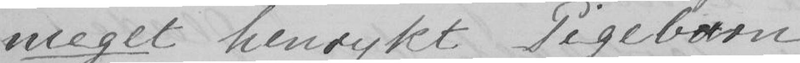meget henrykt Pigebarn,
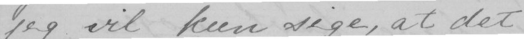jeg vil kun sige, at det
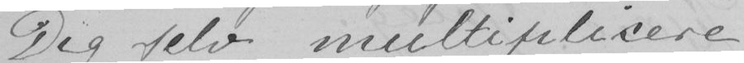Dig selv multiplicere
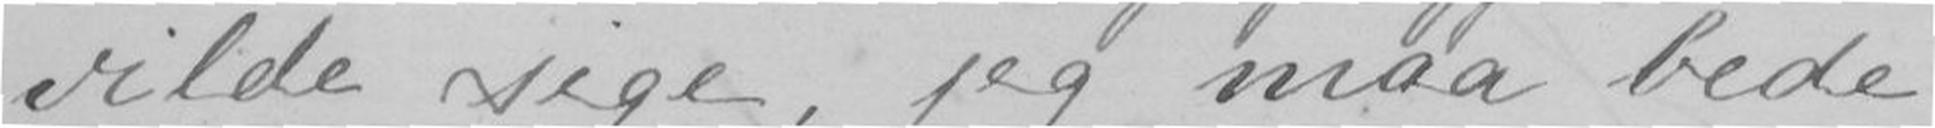vilde sige, jeg maa bede
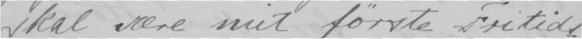skal være mit første Fritids-
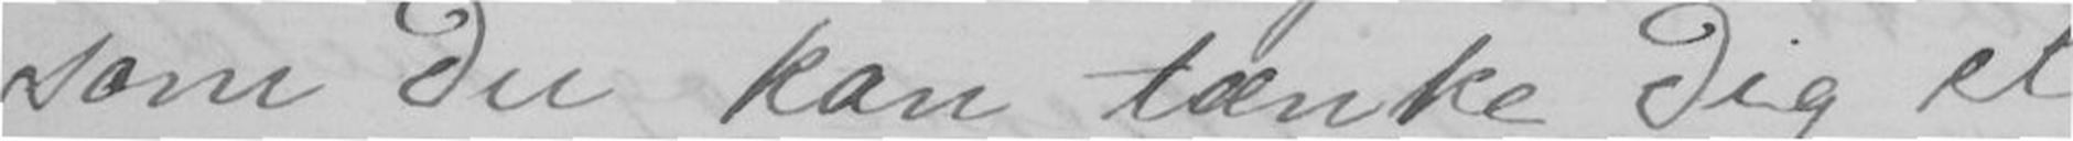som Du kan tænke Dig et
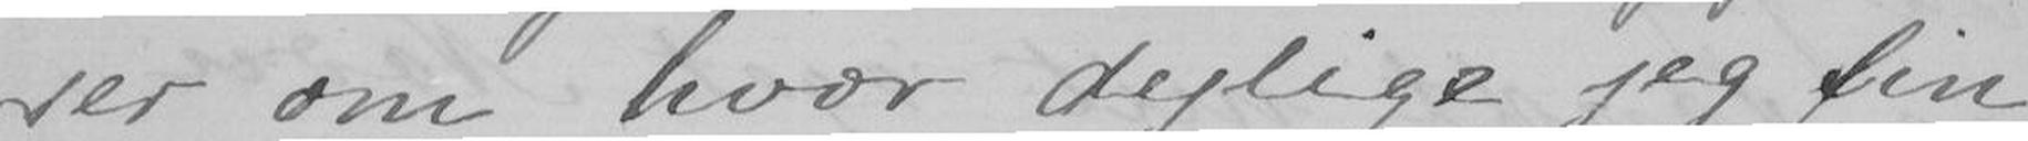ser om hvor dejlige jeg fin-
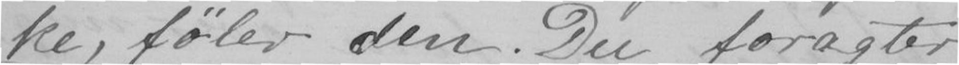ke, føler den. Du foragter
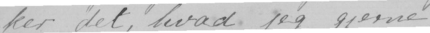ker det, hvad jeg gjerne
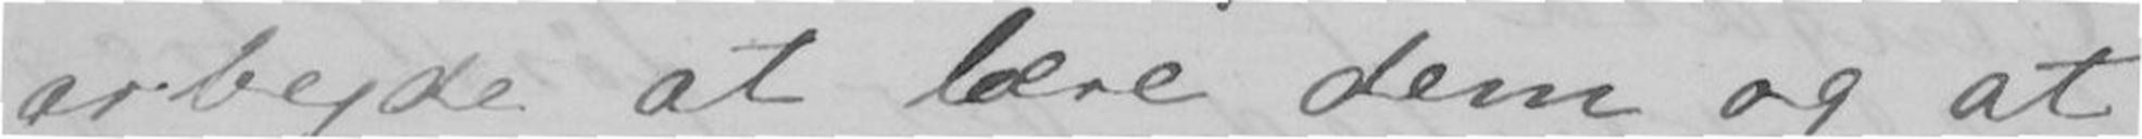arbejde at lære dem og at
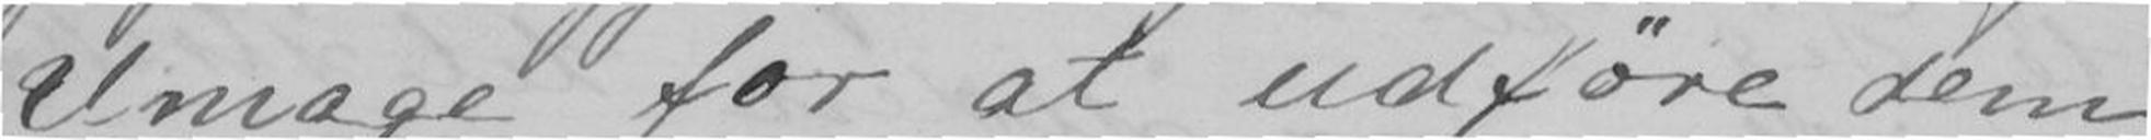Umage for at udføre dem
